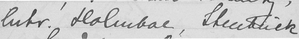lntr. Holmboe, Stenbuck
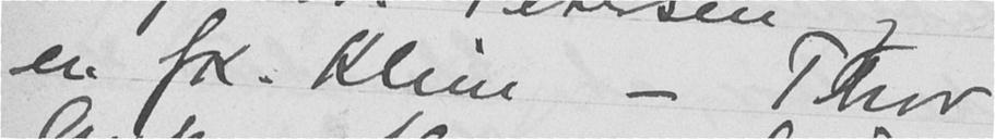en frk. Klein - Thor
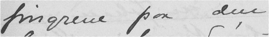fingrene paa den
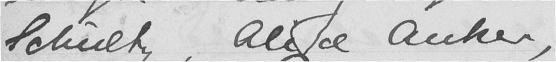Schultz, Alice Anker,
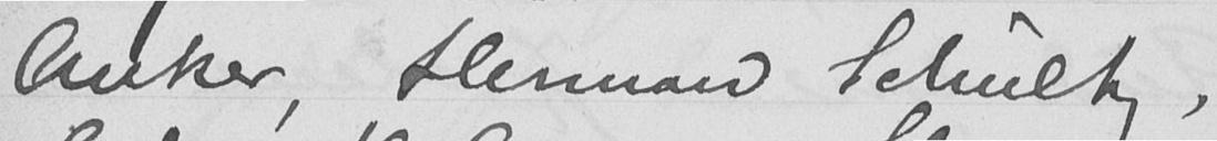Anker, Herman Schultz,
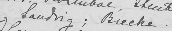og Sandvig; Brecke.
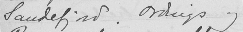Sandefjord. Ordings og
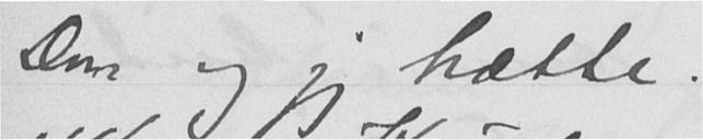Don og jeg trætte.
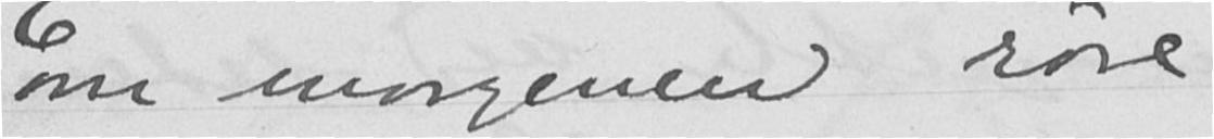om morgenen røre
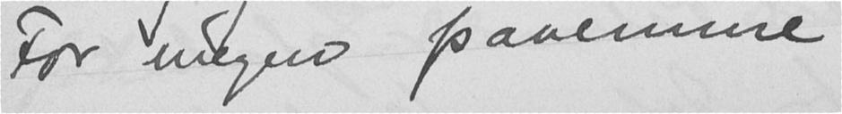For megen pavemine
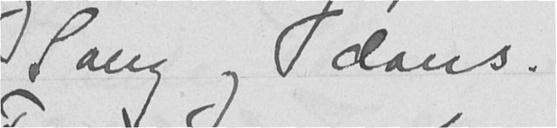Sang og dans.
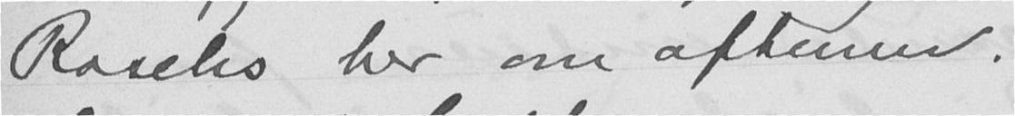Raschs her om aftenen.
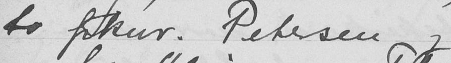to frkner. Petersen og
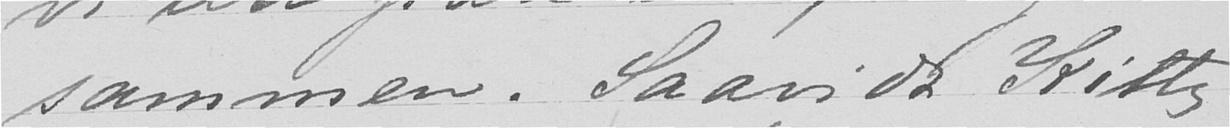sammen. Saavidt Kitty
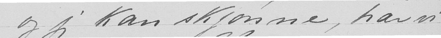og jeg kan skjønne, har vi
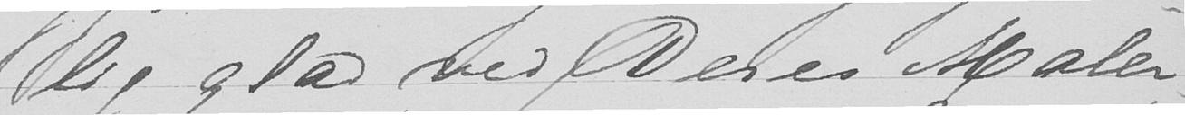lig glad ved Deres Maler-
